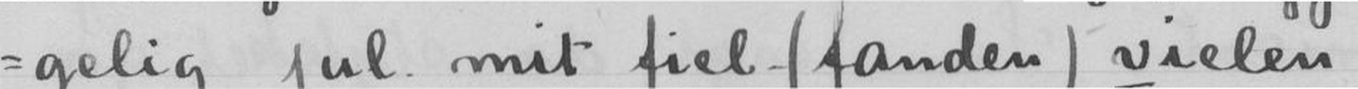gelig jul mit fiel-(fanden) vielen
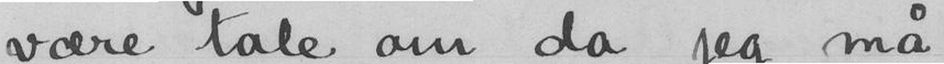være tale om da jeg må
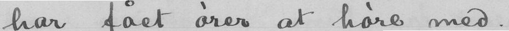har fået ører at høre med.
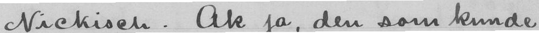Nickisch. Ak ja, den som kunde
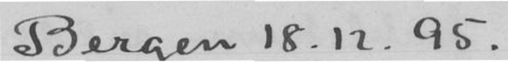Bergen 18.12.95.
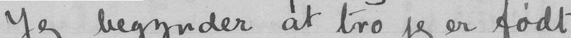Jeg begynder at tro jeg er født
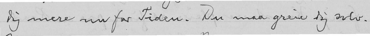dig mere nu for Tiden. Du maa greie dig selv.
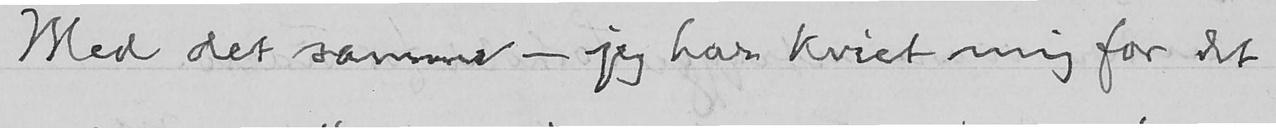Med det samme - jeg har kviet mig for det
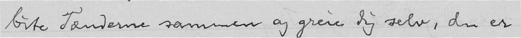bite Tænderne sammen og greie dig selv, du er
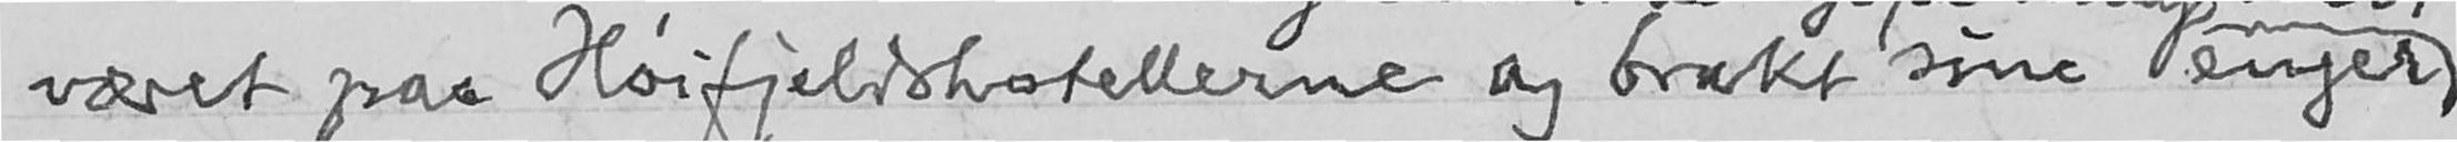været paa Høifjeldshotellerne og brukt sine Penger,
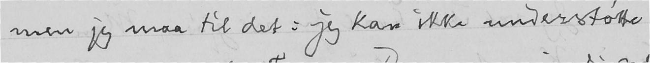men jeg maa til det: jeg kan ikke understøtte
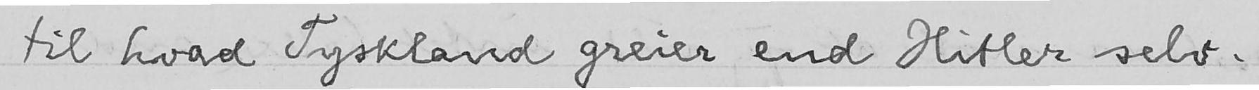til hvad Tyskland greier end Hitler selv.
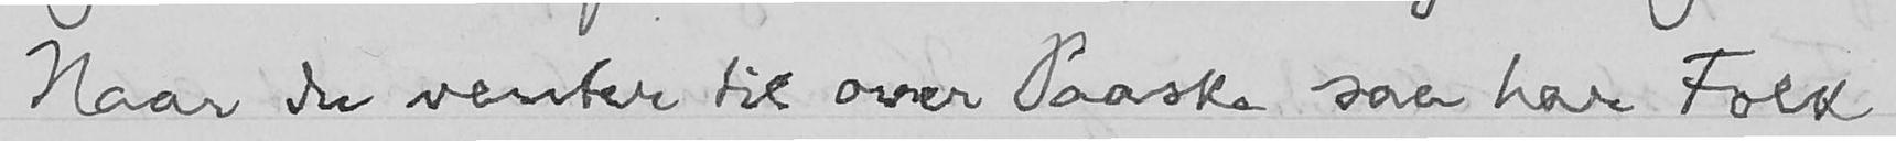Naar du venter til over Paaske saa har Folk
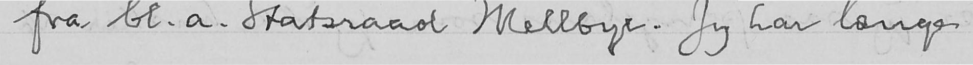fra bl.a. Statsraad Mellbye. Jeg har længe
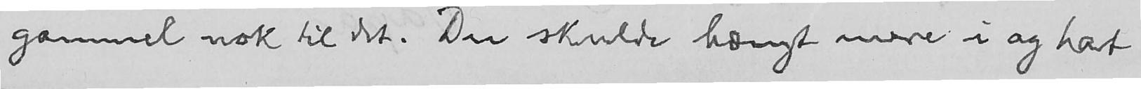gammel nok til det. Du skulde hængt mere i og hat
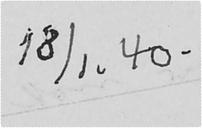18/1. 40.
